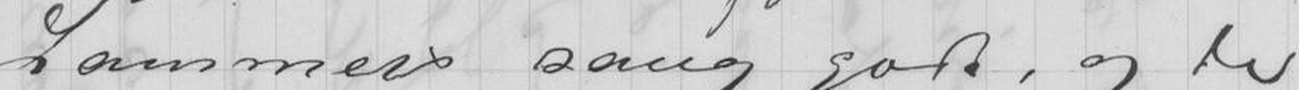Lammers sang godt, og de
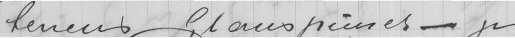tenens Glanspunct - jeg
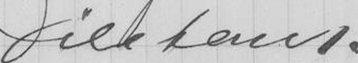Diletant.
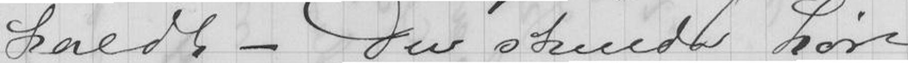kaldt - Du skulde høre
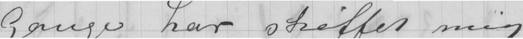Gange har skaffet mig
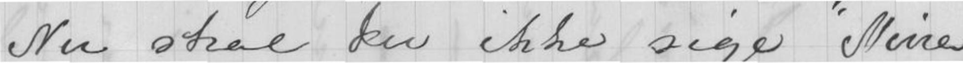Nu skal Du ikke sige "Nine
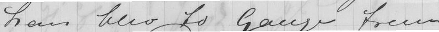- han blev to Gange frem-
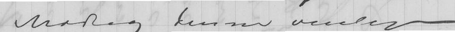Modtag denne venlige
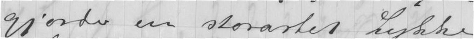gjorde en storartet Lykke
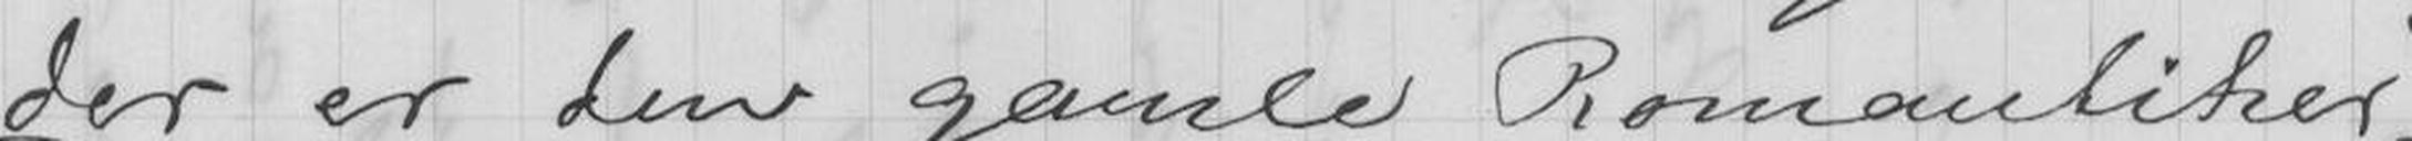der er den gamle Romantiker"!
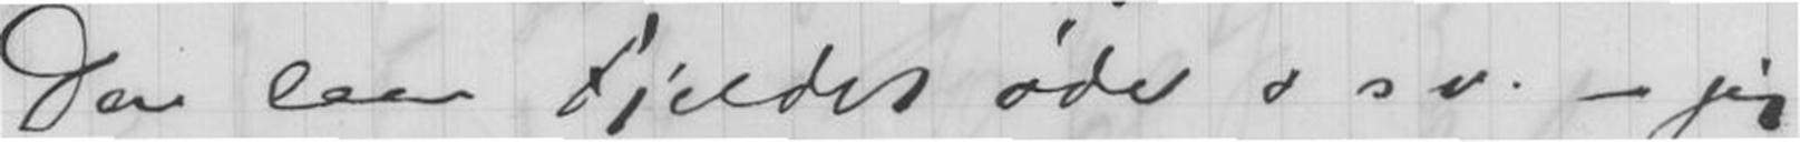"Der laa Fjeldet øde o s v. - jeg
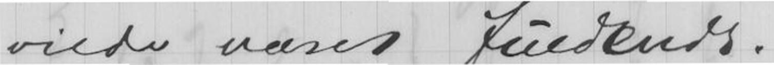vilde været fuldendt.
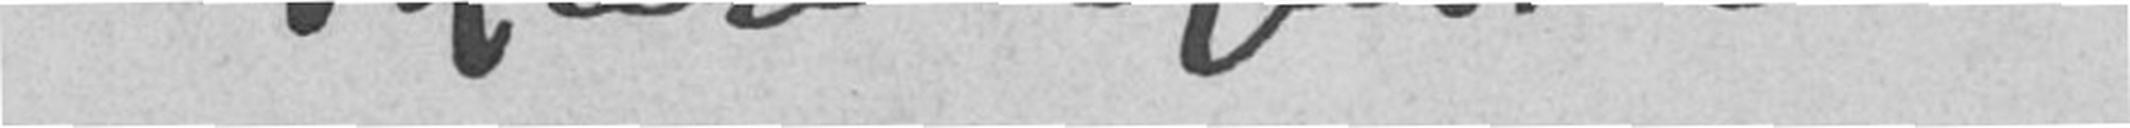Kjære Gustav
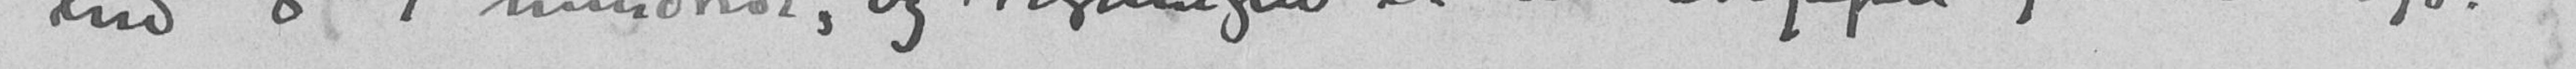end 8 - 9 hundrede, og tegningen er nu stoppet ganske op.
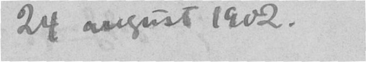24 august 1902.
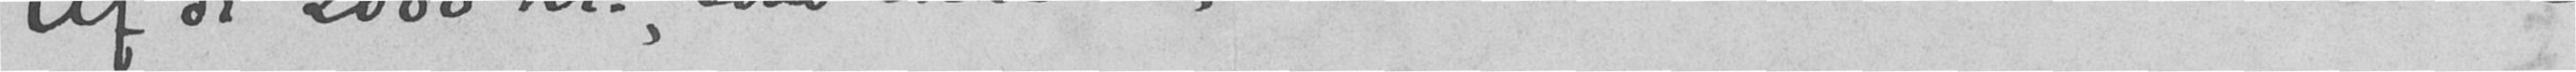Af de 2000 kr., som skal til, er der ikke indkommet mere
